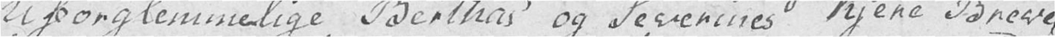uforglemmelige Berthas' og Severines' Kjere Breve,
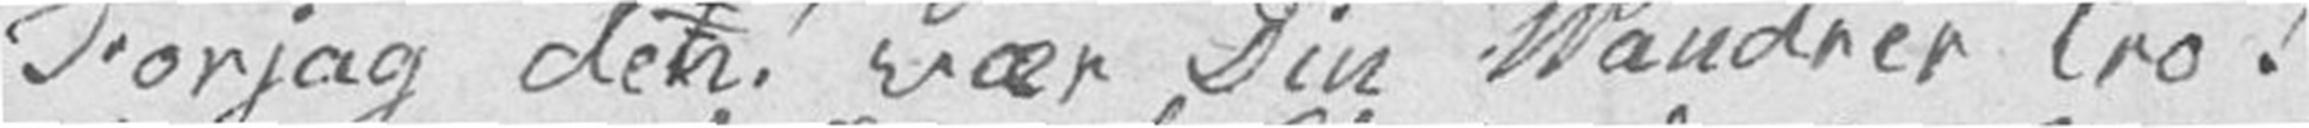Forjag den! vær Din Vandrer tro!
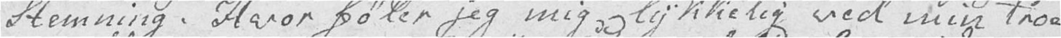Stemning. Hvor føler jeg mig lykkelig ved min troe
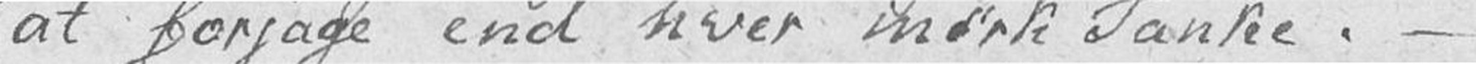at forjage end hver mørk Tanke. –
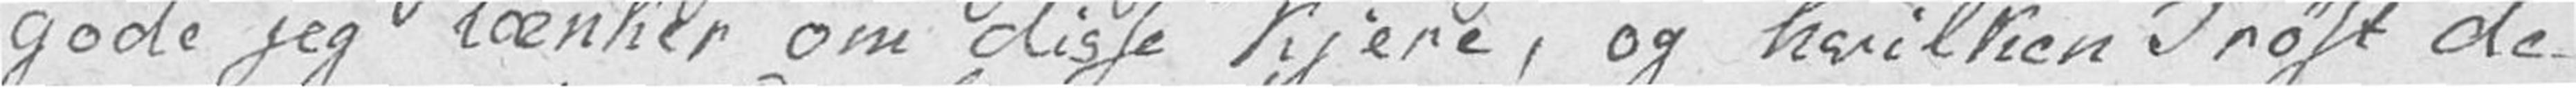gode jeg tænker om disse kjere, og hvilken Trøst de
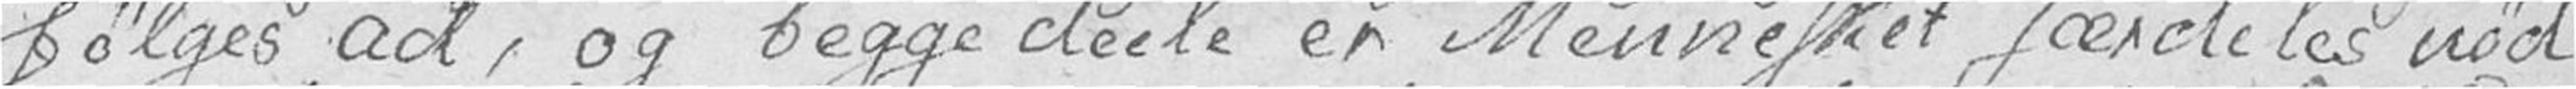følges ad, og begge deele er Mennesket særdeles nød
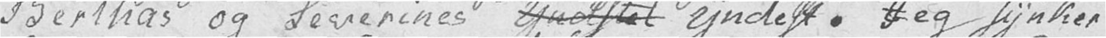Berthas' og Severines' yndstet yndest. Jeg synker
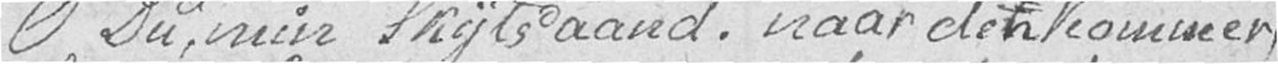O Du, min Skytsaand! naar den kommer,
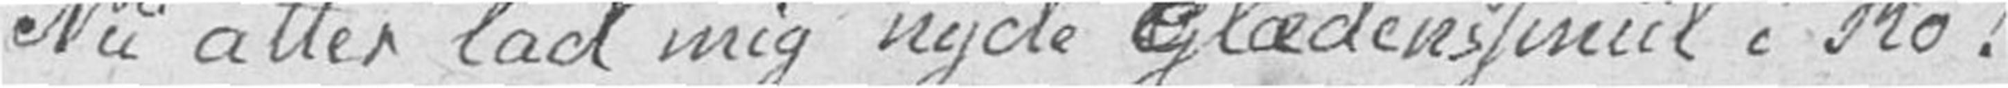Nu atter lad mig nyde Glædenssmiil i Ro!
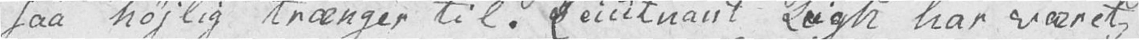saa højlig trænger til. Leiutnant Leigh har været
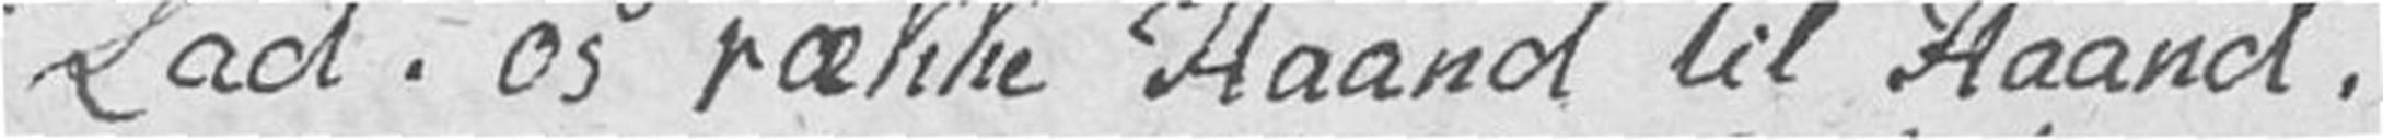Lad! os række Haand til Haand!
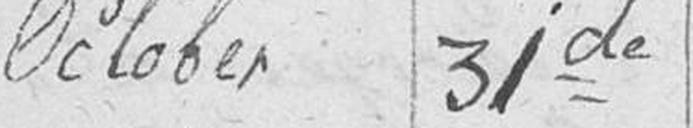October 31de
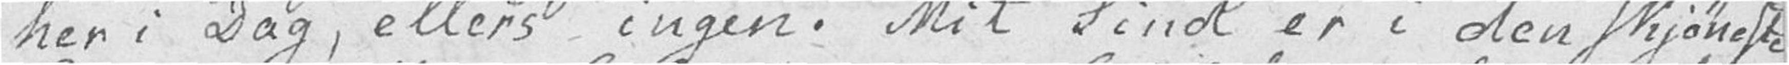her i Dag, ellers ingen. Mit Sind er i den skjøneste
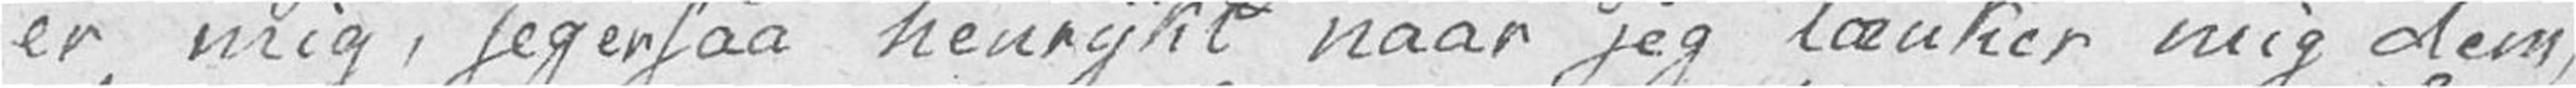er mig, jeg er saa henrykt naar jeg tænker mig dem,
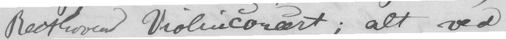Beethovens Violinconcert; alt ved
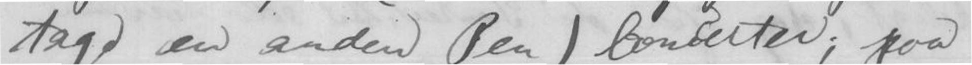tage en anden Pen) Concerter; paa
